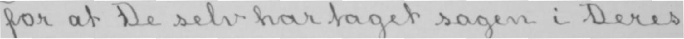for at De selv har taget sagen i Deres
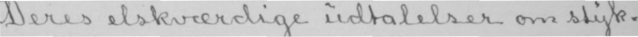Deres elskværdige udtalelser om styk-
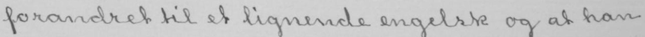forandret til et lignende engelsk og at han
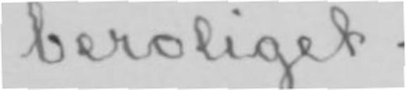beroliget.-
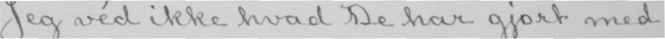Jeg véd ikke hvad De har gjort med
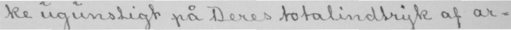ke ugunstigt på Deres totalindtryk af ar-
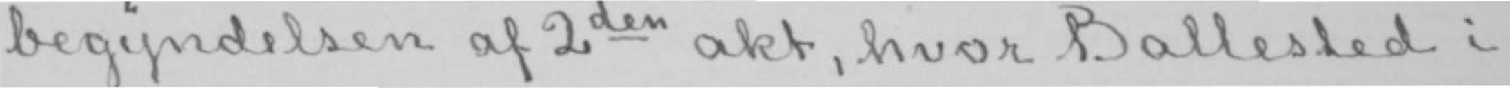begyndelsen af 2den akt, hvor Ballested i
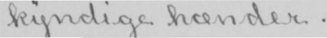kyndige hænder.
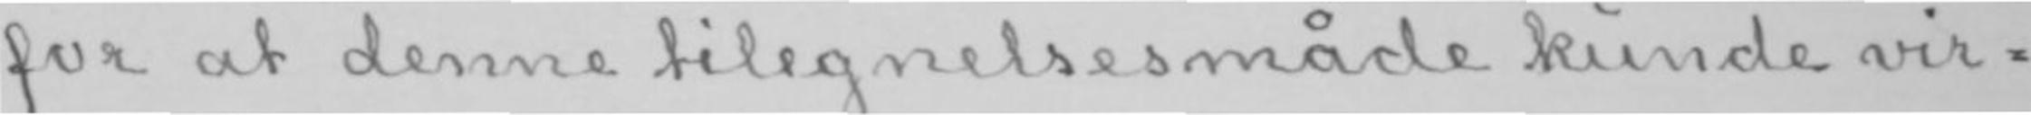for at denne tilegnelsesmåde kunde vir-
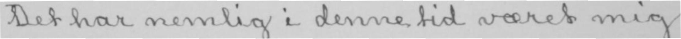Det har nemlig i denne tid været mig
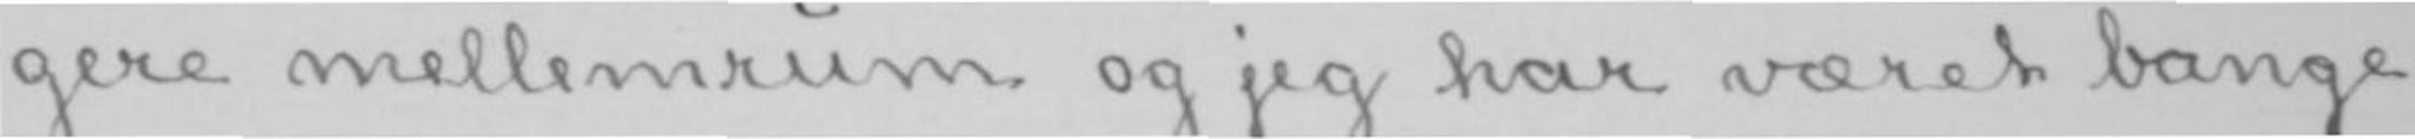gere mellemrum og jeg har været bange
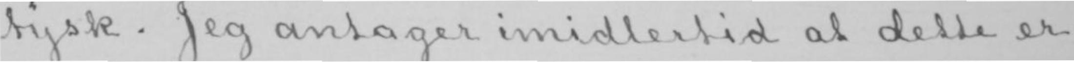tysk. Jeg antager imidlertid at dette er
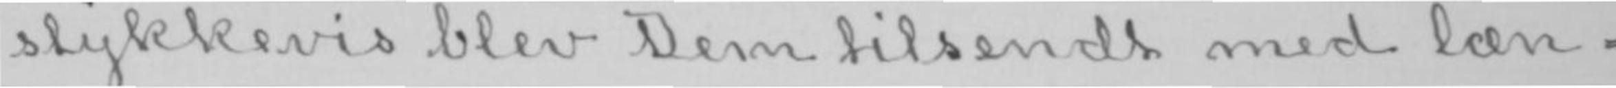stykkevis blev Dem tilsendt med læn-
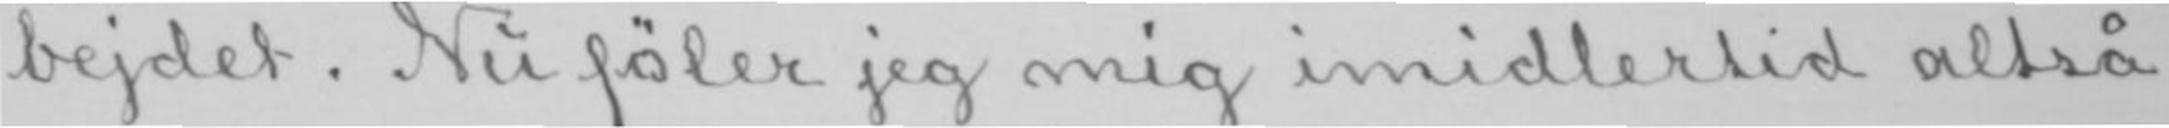bejdet. Nu føler jeg mig imidlertid altså
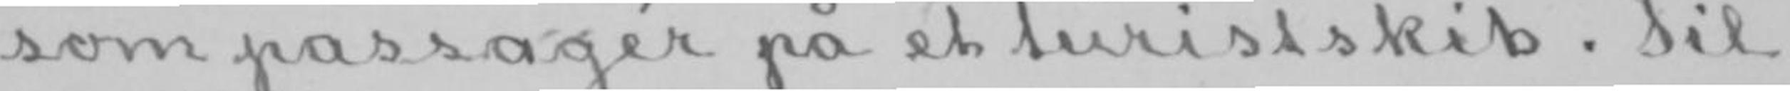som passagér på et turistskib. Til
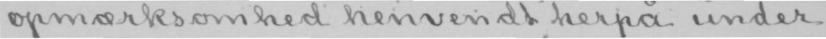opmærksomhed henvendt herpå under
